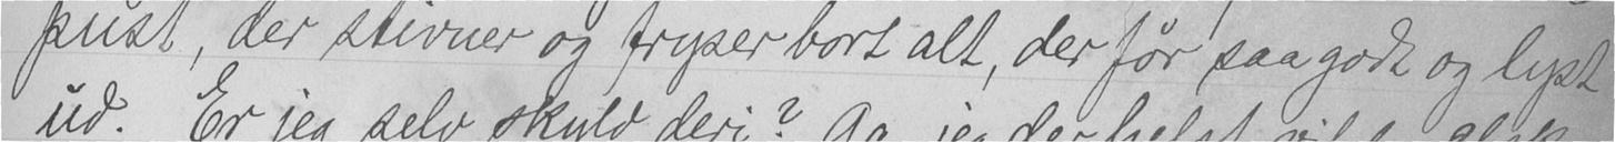pust, der stivner og fryser bort alt, der før saa godt og lyst
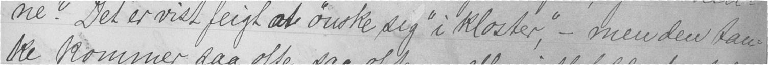ne? Det er vist feigt at ønske sig "i kloster," - men den tan-
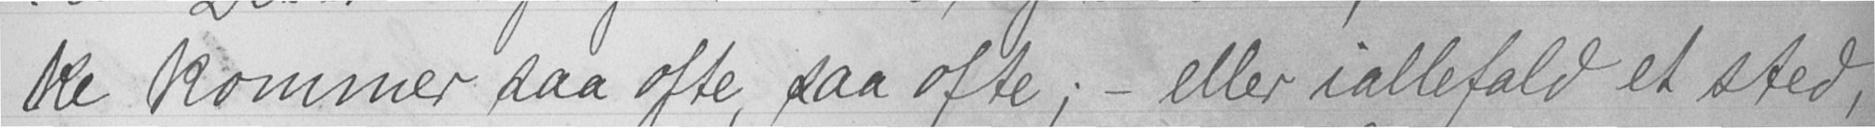ke kommer saa ofte, saa ofte; - eller iallefald et sted,
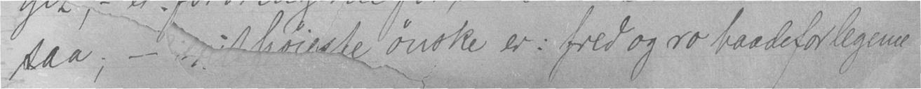saa; - mit høieste ønske er: fred og ro baade for legeme
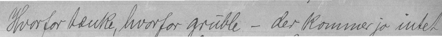Hvorfor tænke, hvorfor gruble - der kommer jo intet
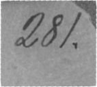281.
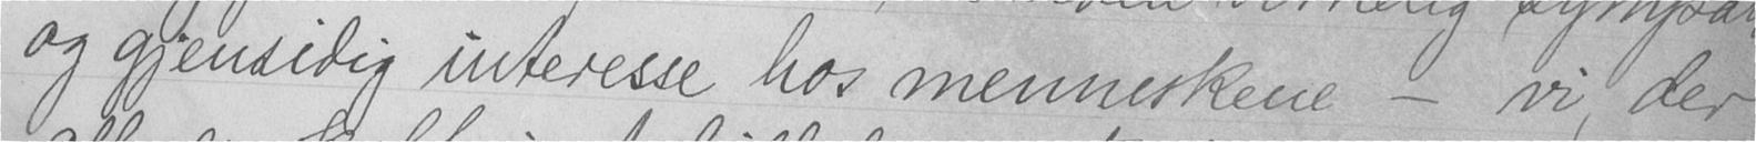og gjensidig interesse hos menneskene - vi, der
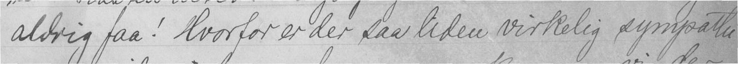aldrig faa! Hvorfor er der saa liden virkelig sympathi
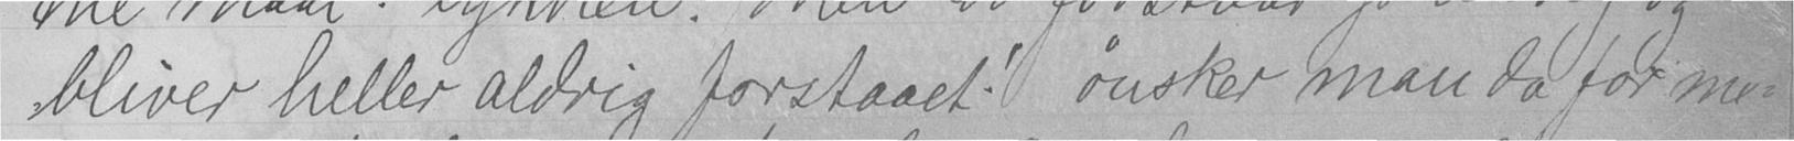bliver heller aldrig forstaaet! ønsker man da for me-
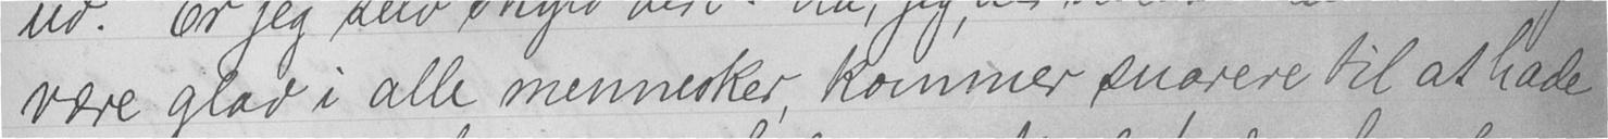være glad i alle mennesker, kommer snarere til at hade
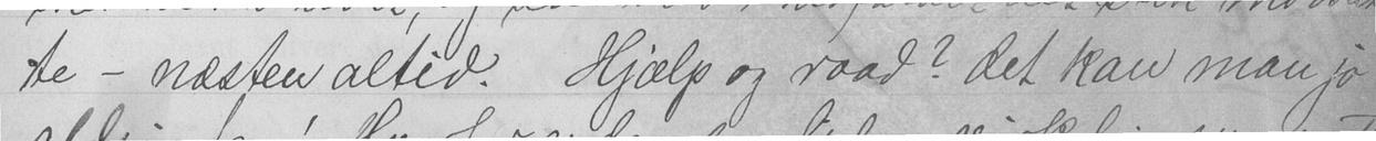te - næsten altid. Hjælp og raad? det kan man jo
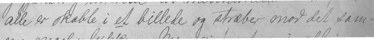alle er skabte i et billede og stræber mod det sam-
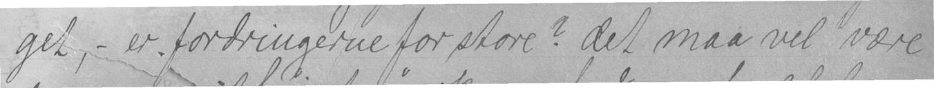get, - er fordringerne for store? det maa vel være
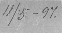11/5-97.
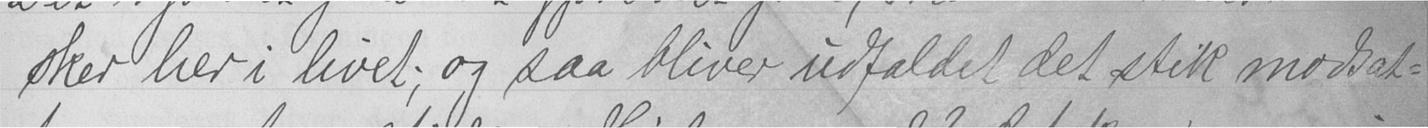sker her i livet; og saa bliver udfaldet det stik modsat-
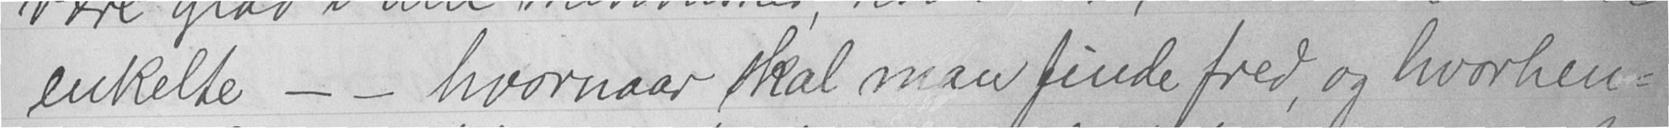enkelte - - hvornaar skal man finde fred, og hvorhen-
